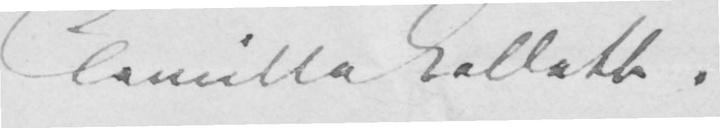Camilla Collett.
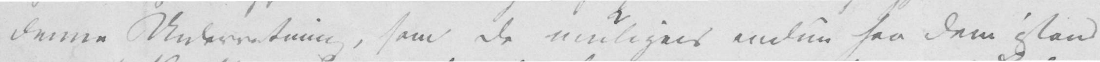denne Underretning, som De muligens endnu saa Dem istand
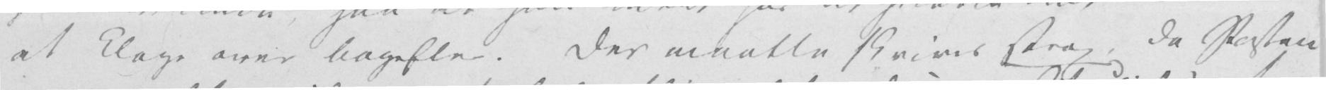at klage over bagefter. Der maatte skrives strax, da Posten
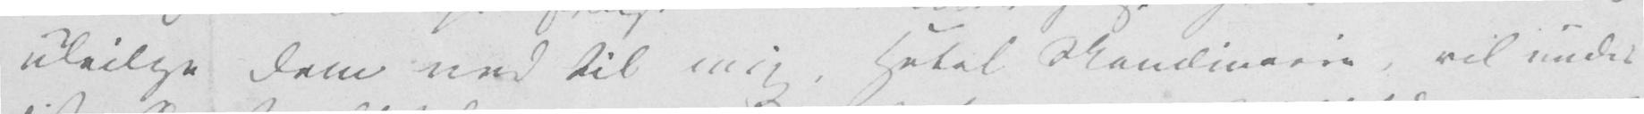uleilige Dem ned til mig, Hotel Skandinavie, vil under
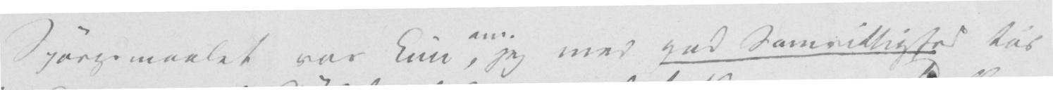Spørgsmaalet var kun, jeg med god Samvittighed tør
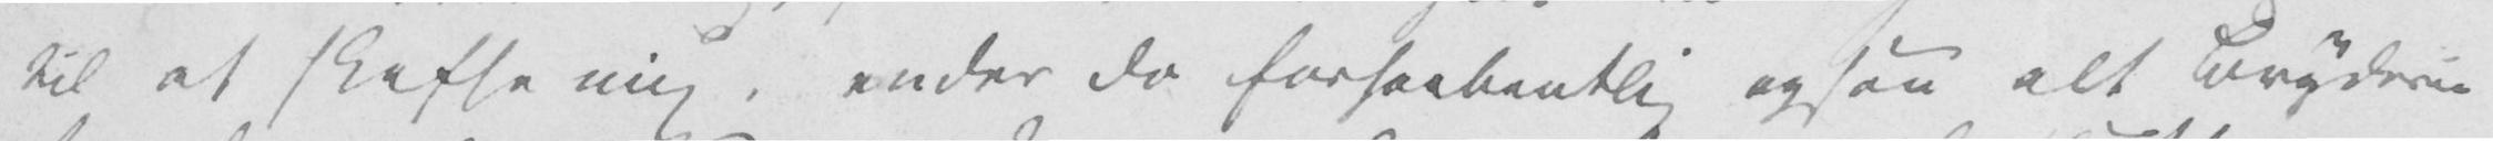til at skaffe mig, ender da forhaabentlig ogsaa alt Bryderie
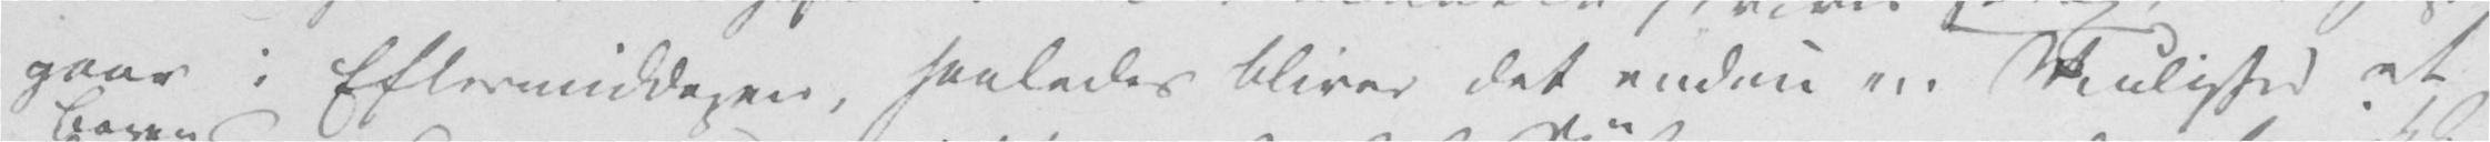gaar i Eftermiddagen, saaledes bliver det endnu en Mulighed at
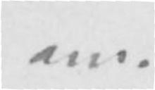om
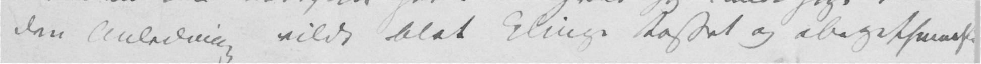den Anledning vilde blot klinge tosset og abgeschmacht
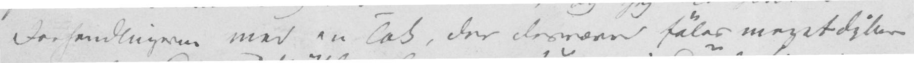Forhandlingerne med en Tak, der desværre føles meget dybere
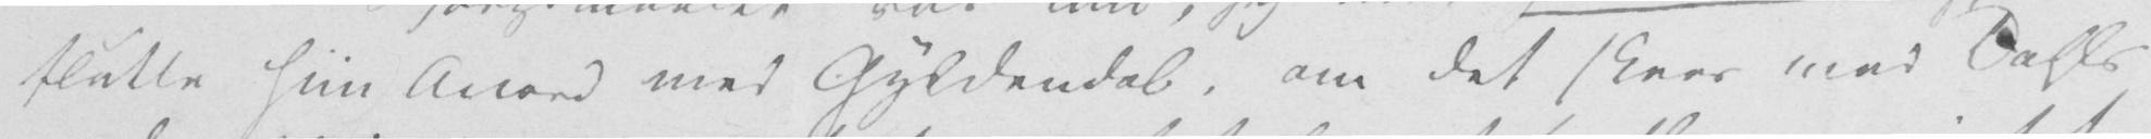slutte hiin Accord med Gyldendal, om det skeer med Dahls
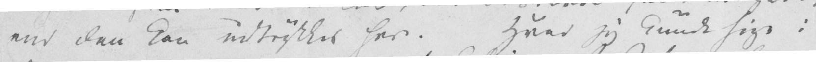end den kan udtrykkes her. Hvad jeg kunde sige i
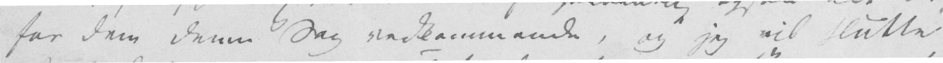for Dem denne Sag vedkommende, og jeg vil slutte
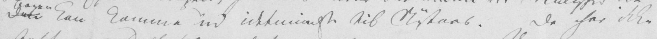den kan komme ud idetmindste til Nytaar. De har ikke
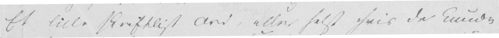Et lille skriftligt Ord, eller helst hvis De kunde
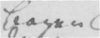Bogen
